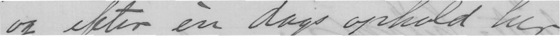og efter èn dags ophold her
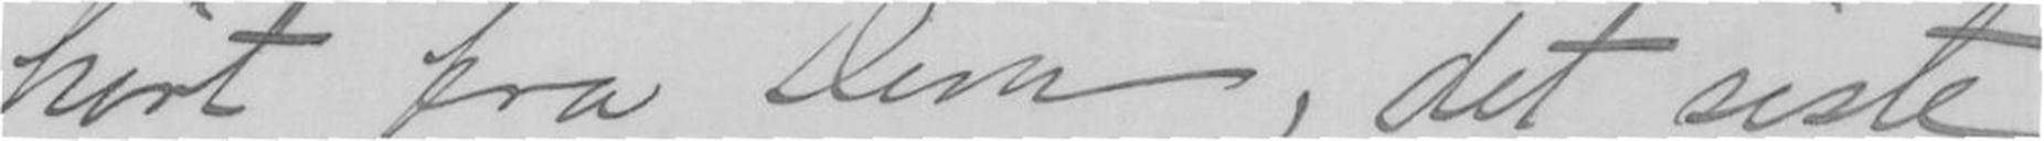hørt fra Dem, det siste
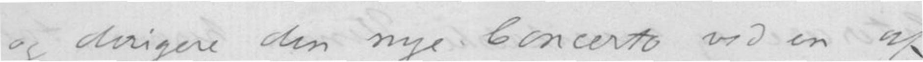og derigere den nye Concerto ved en af
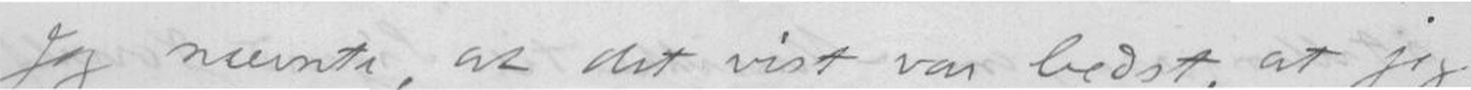Jeg nævnte, at det vist var bedst, at jeg
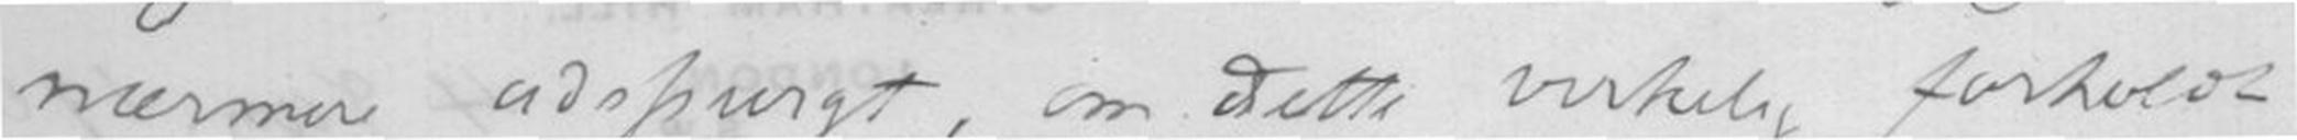nærmere udspurgt, om dette virkelig forholdt
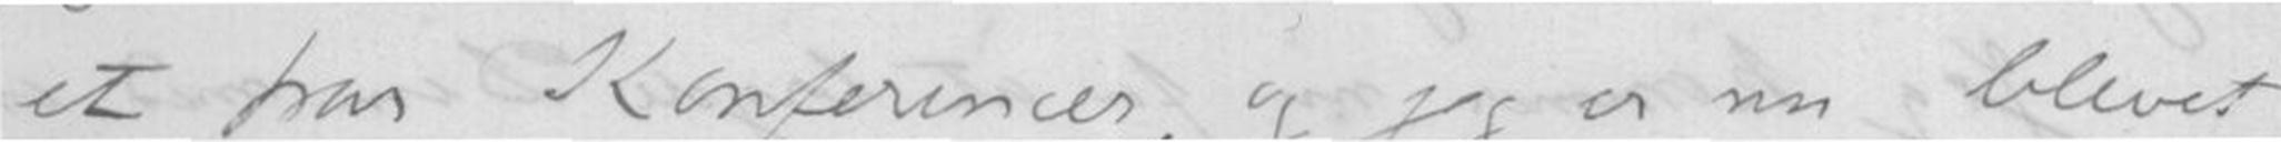et par Konferencer, og jeg er nu blevet
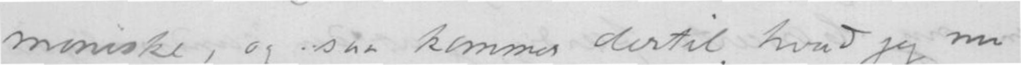moniske, og saa kommer dertil, hvad jeg nu
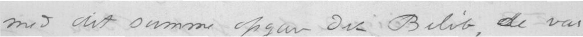med det samme opgav Dit Beløb, de var
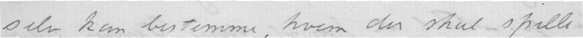selv kan bestemme, hvem der skal spille
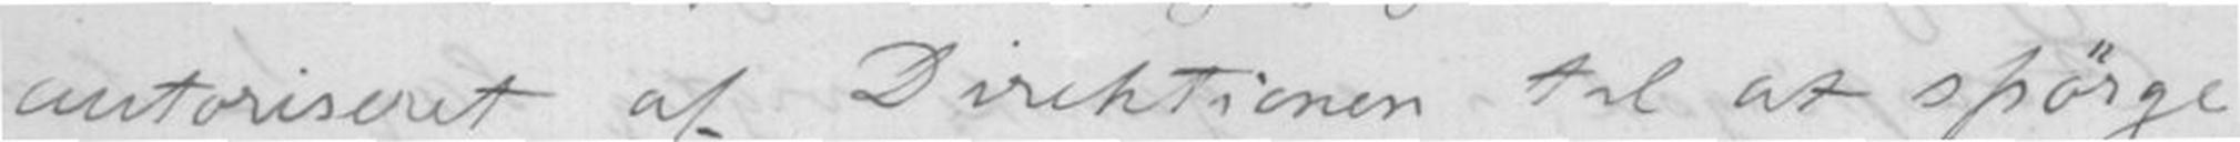autoriseret af Direktionen til at spørge
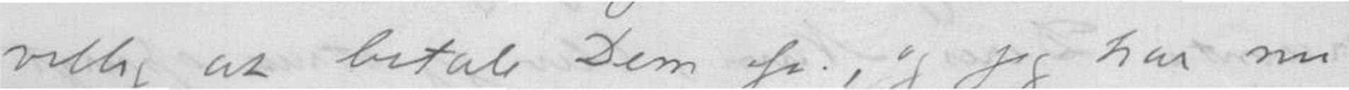villig at betale Dem osv., og jeg har nu
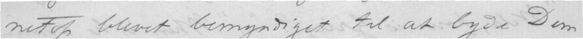netop blevet bemyndiget til at byde Dem
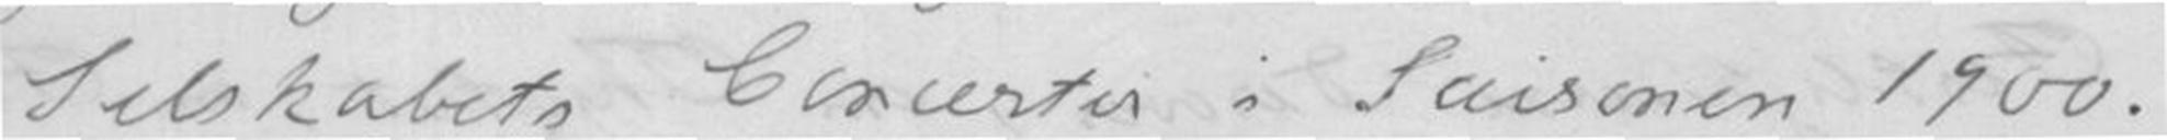Selskabets Concerter i Saisonen 1900.
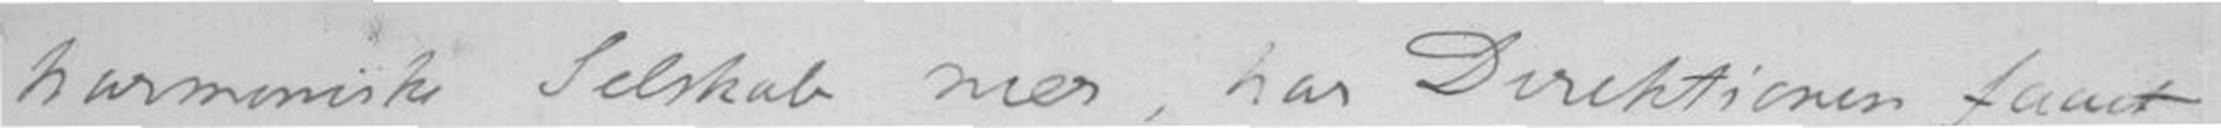harmoniske Selskab nær, har Direktionen faaet
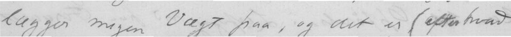lægger megen Vægt paa, og det er (efter hvad
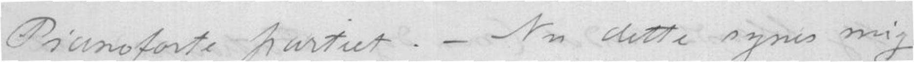Pianoforte partiet. - Nu dette synes mig
